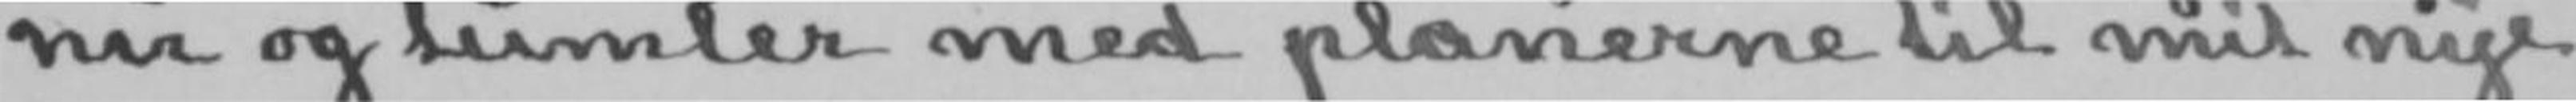nu og tumler med planerne til mit nye
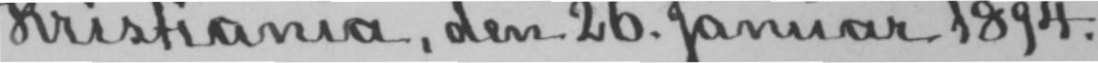Kristiania, den 26. Januar 1894.
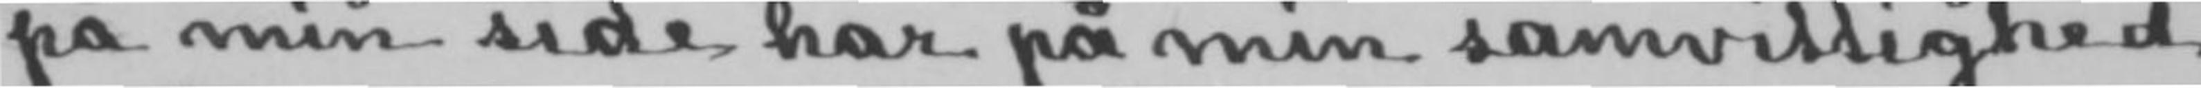på min side har på min samvittighed
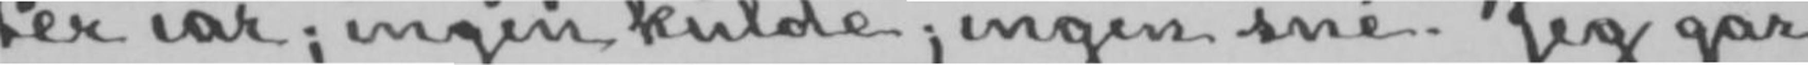ter iår; ingen kulde, ingen sné. Jeg går
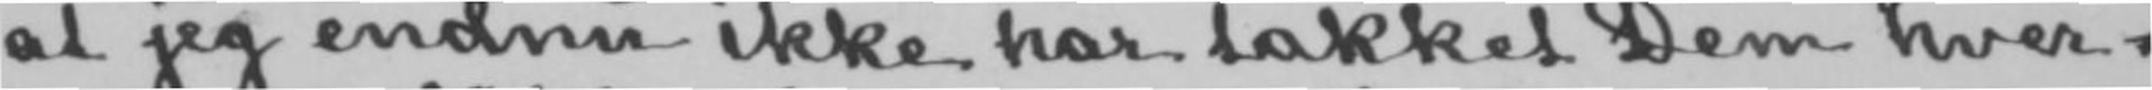at jeg endnu ikke har takket Dem hver-
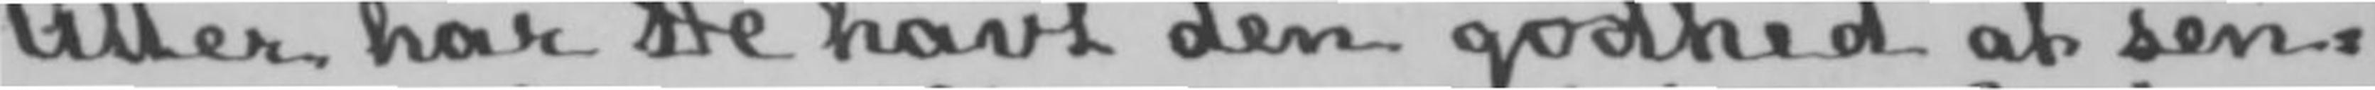Atter har De havt den godhed at sen-
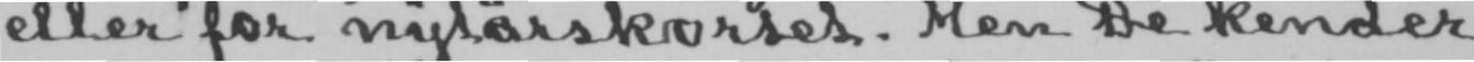eller for nytårskortet. Men De kender
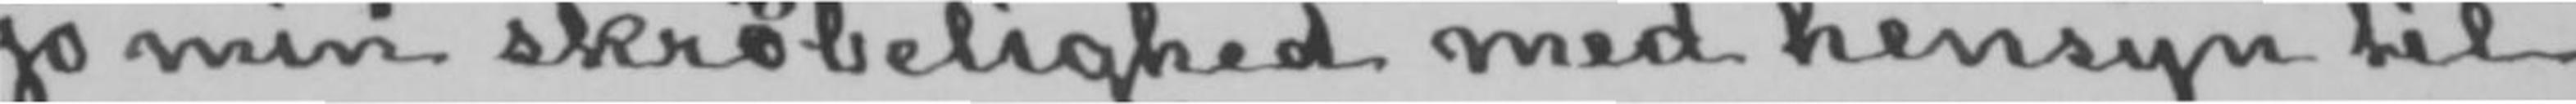jo min skrøbelighed med hensyn til
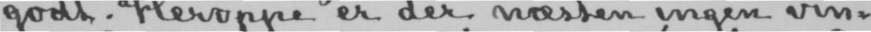godt. Heroppe er der næsten ingen vin-
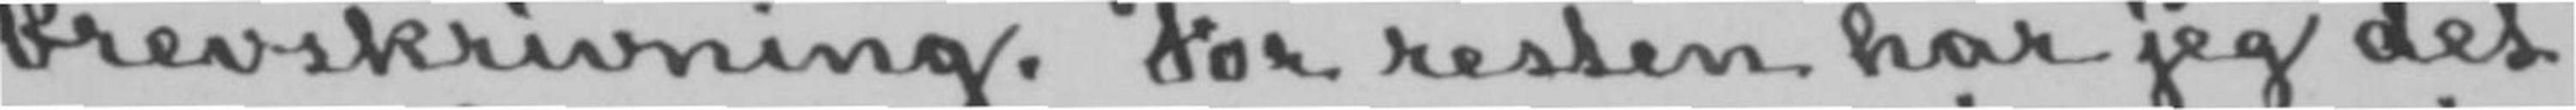brevskrivning. For resten har jeg det
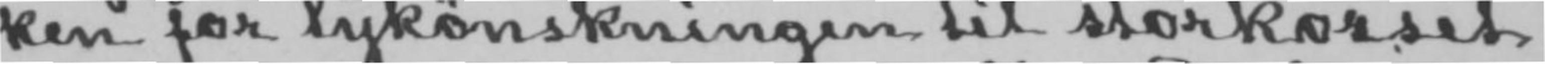ken for lykønskningen til storkorset
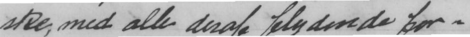ske, med alle deraf flydende for-
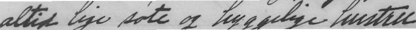altid lige søte og hyggelige hustru
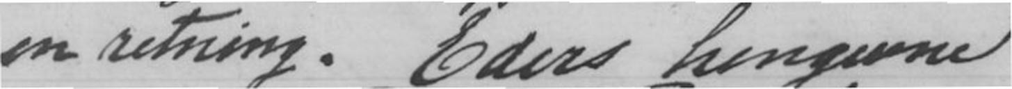en retning.Eders hengivne
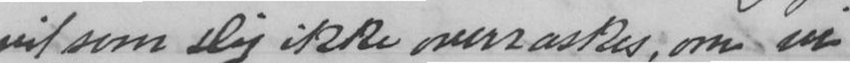vil som Dig ikke overraskes, om vi
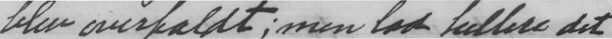blev overfaldt; men lad hellere det
